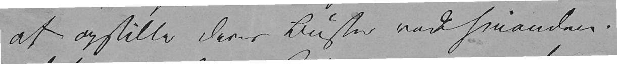at opstille deres Buster ved hinanden.
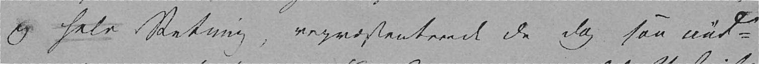og hele Retning, repræsenterede de dog saa nød-
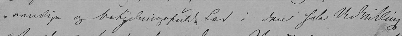vendige og betydningsfulde Led i den hele Udvikling,
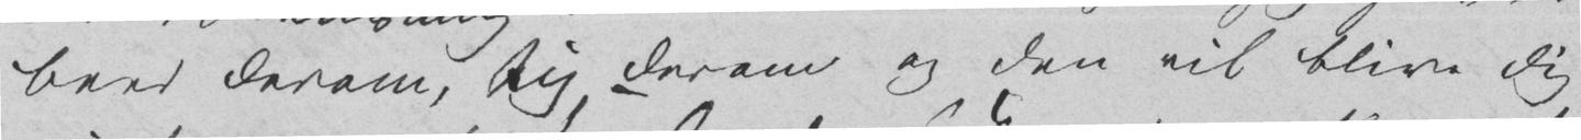beed derom, tig derom og den vil blive Dig
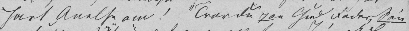havt Anelse om! «Tror Du paa Gud Fader, Søn
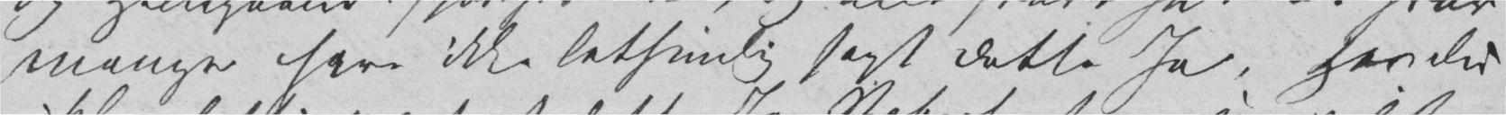mange have ikke letsindig sagt dette Ja, Har Du
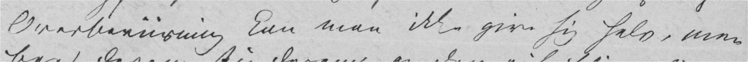Overbeviisning kan man ikke give sig selv, men
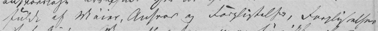fuldt af Møier, Ansvar og Forpligtelser, Forpligtelser
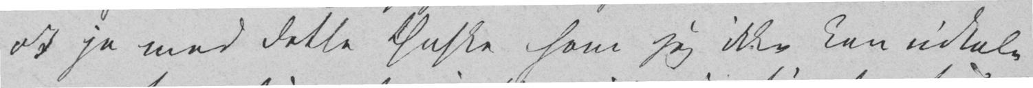ak ja med dette Ønske som jeg ikke kan udtale
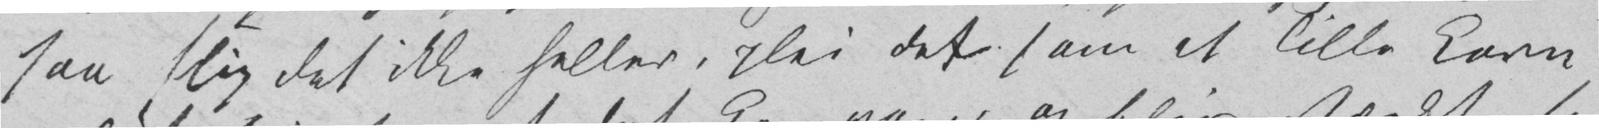saa slip det ikke heller, plei det som et lille Korn
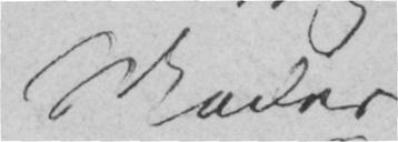Moder
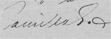Camilla C.
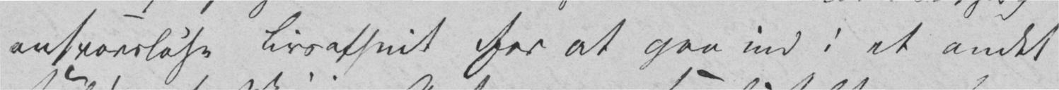ansvarsløse Livsafsnit for at gaa ind i et andet
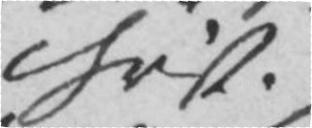christ.
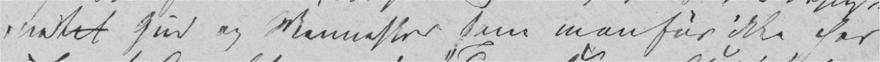mod til Gud og Mennesker som man før ikke har
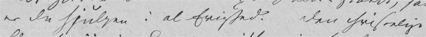er Du hjulpen i al Evighed. Den christelige
